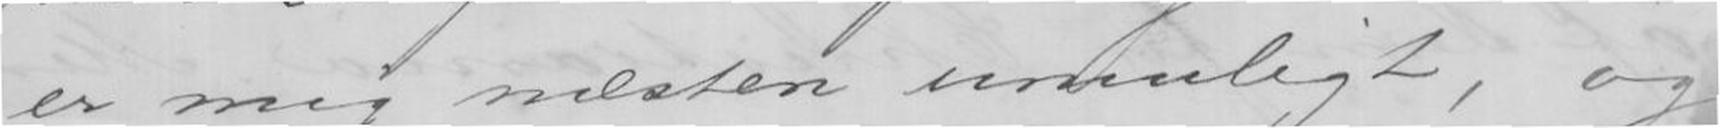er mig nædsten umuligt, og
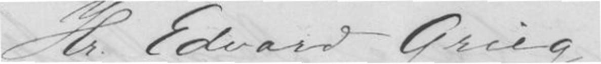Hr. Edvard Grieg!
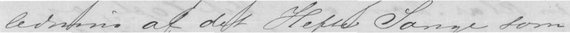ledning af det Hefte Sange, som
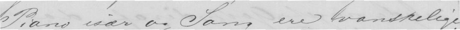Piano især og Sang ere vanskelige,
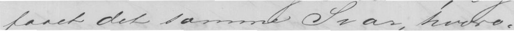faaet det samme svar hvoro-
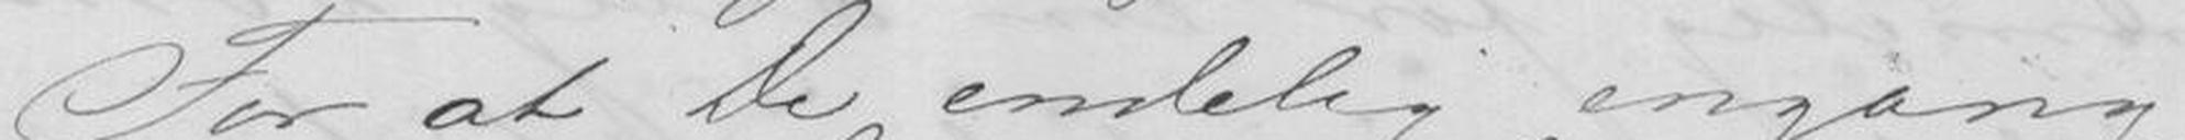For at De endelig engang
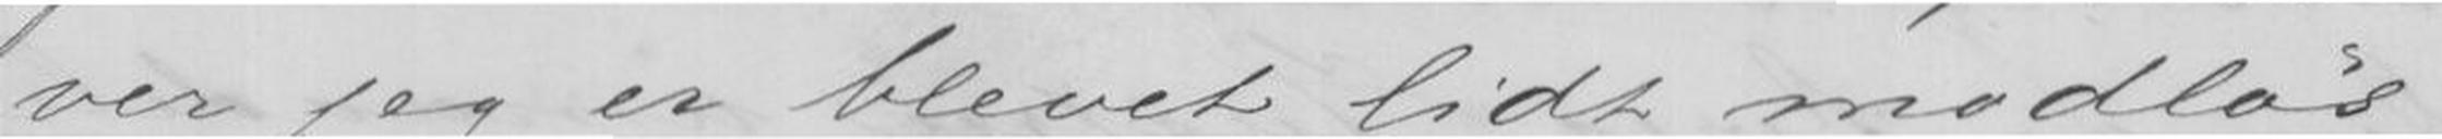ver jeg er blevet lidt modløs;
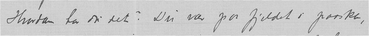Hvordan har du det? Du var paa fjeldet i paasken,
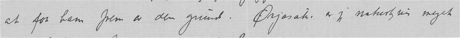at faa ham frem av den grund. Ørjasæter er jeg naturligvis meget
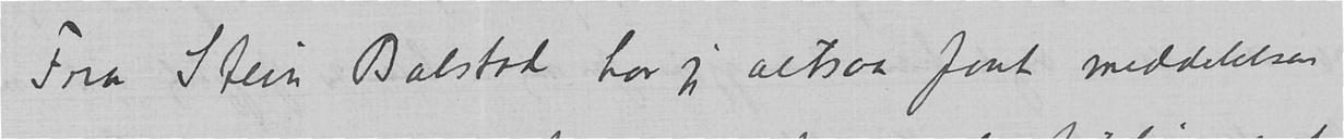Fra Stein Balstad har jeg altsaa faat meddelelsen
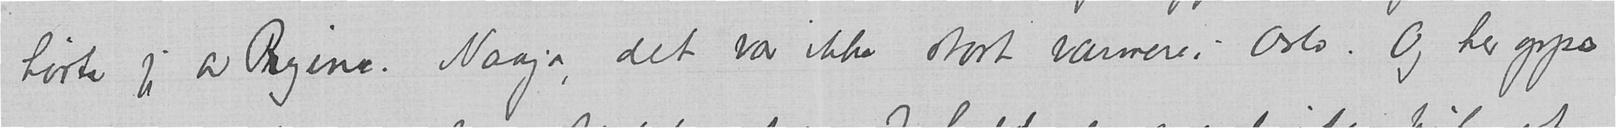hørte jeg av Regine. Naaja, det var ikke stort varmere i Oslo. Og her oppe
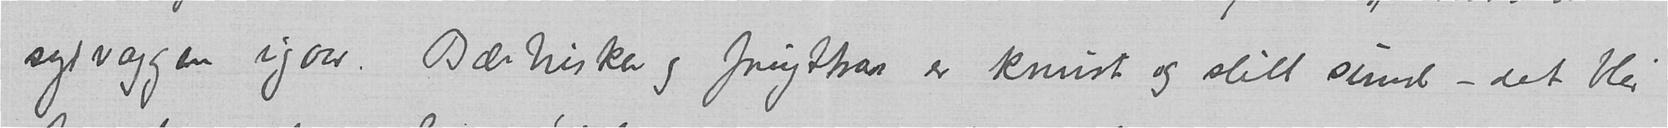sydtvæggen igaar. Bærbusker og frugttrær er knust og slitt sund – det blir
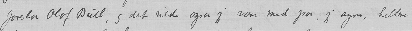foreslaa Olaf Bull, og det vilde ogsaa jeg være med paa, jeg synes, hellere
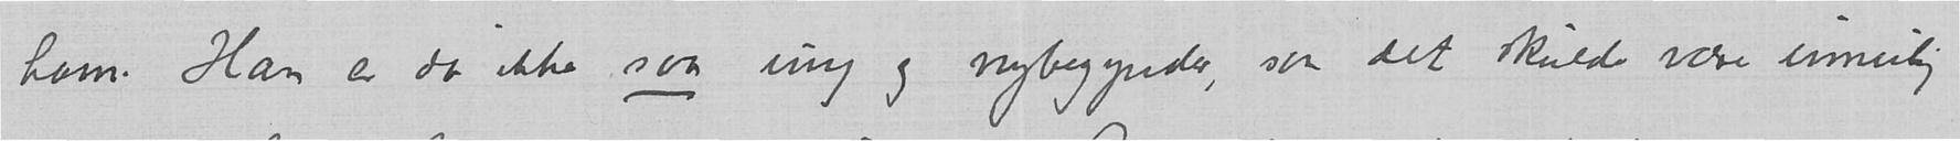ham. Han er da ikke saa ung og nybegynder, saa det skulde være umulig
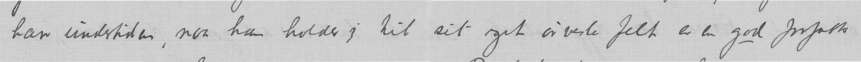han undertiden, naar han holder sig til sit eget ørvesle felt er en god forfatter
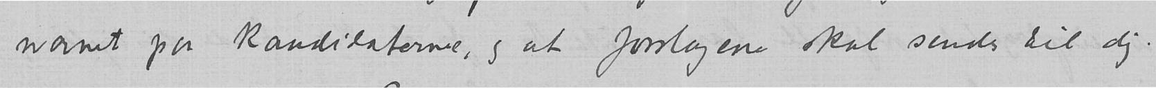navnet paa kandidaterne, og at forslagene skal sendes til dig.
